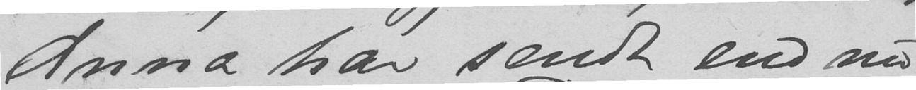Anna har sendt endnu
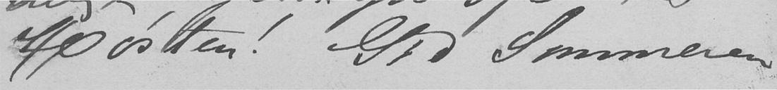Hosten. Gid Sommeren
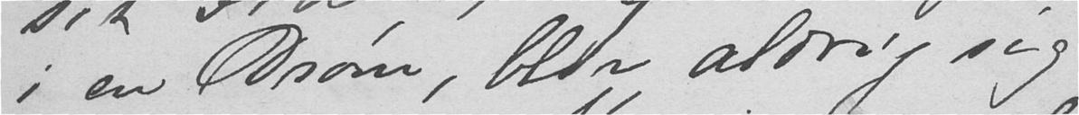i en Drøm, blir aldrig sig
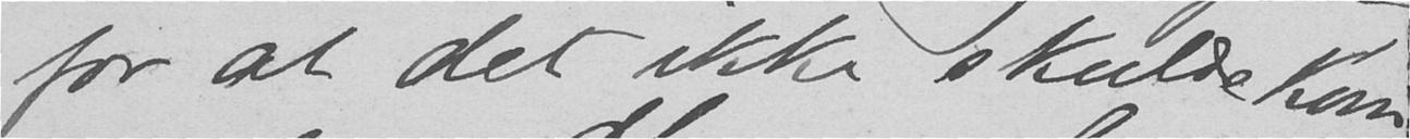for at det ikke skulde kom-
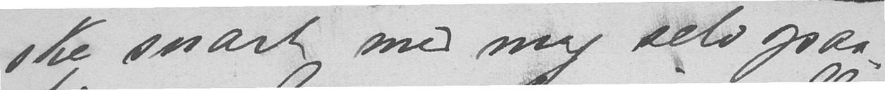ske snart med mig selv ogsaa
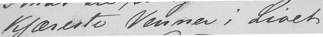kjæreste Venner i Livet
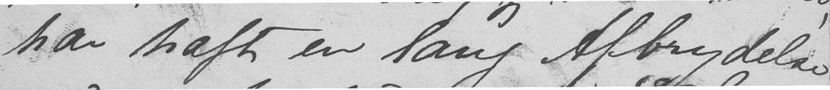har haft en lang Afbrydelse
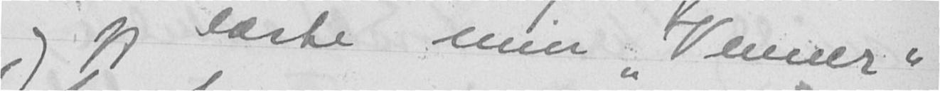og jeg læste min "Venner"
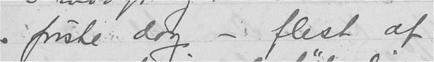første dag - flest af
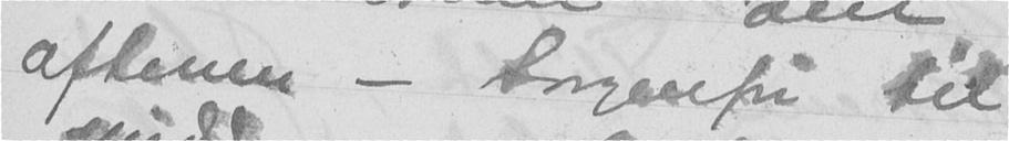aftenen - Sorgenfri til
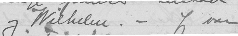og Wilhelm. - Jeg var
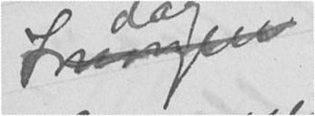Imorgen
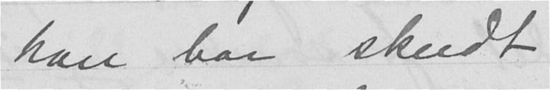han har skudt
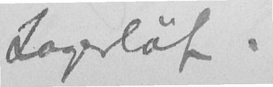Lagerløf.
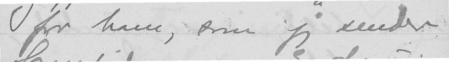for ham, som jeg sender
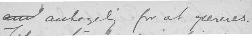and antagelig for at opereres.
